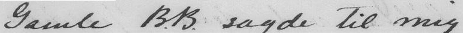Gamle B.B. sagde til mig
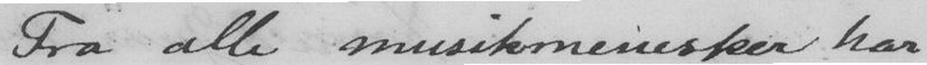Fra alle musikmennesker har
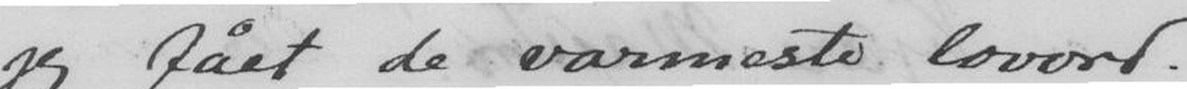jeg fået de varmeste lovord.
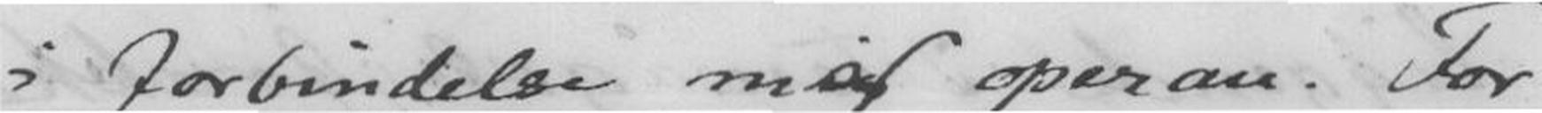i forbindelse med operan. For
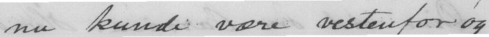nu kunde være vestenfor og
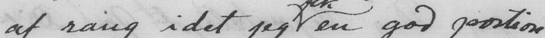af rang idet jeg en god portion
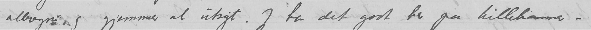allevegne og gjemmer al utsigt.  Jeg har det godt her paa Lillehammer –
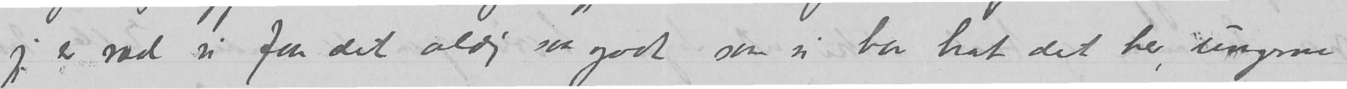jeg er ræd vi faar det aldrig saa godt som vi har hat det her, ungerne
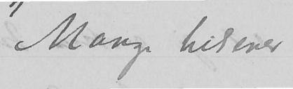Mange hilsener
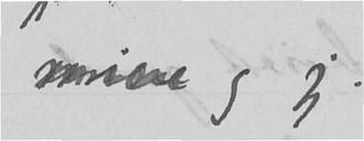mine og jeg.
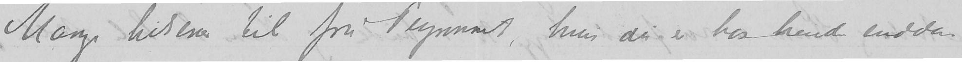Mange hilsener til fru Peynnert??, hvis du er hos hende endda.
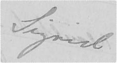Sigrid
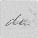din
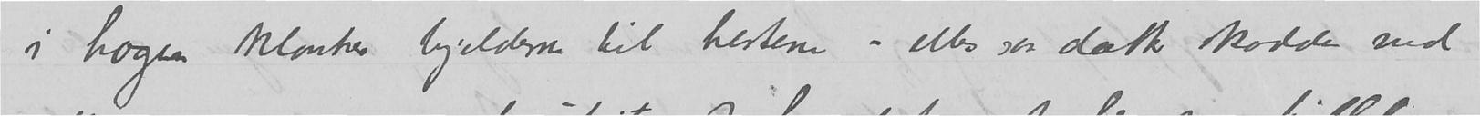i hagen/hogen klonker bjelderne til hestene – ellers saa dætter skodder ned
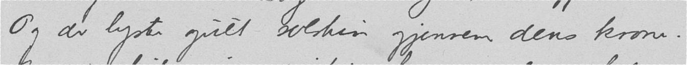Og der lyste gutt solskin gjennem dens krone.
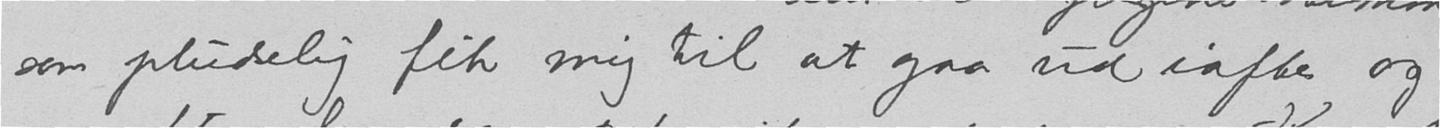som pludselig fik mig til at gaa ud iaftes og
